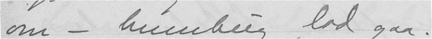om - humbug, lad gaa.
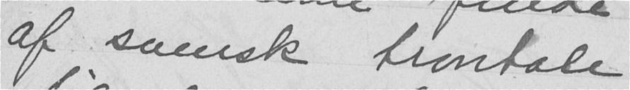af svensk trontale
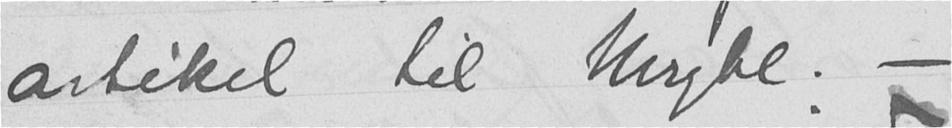artikel til Mrgbl. -
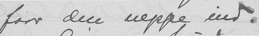faar den neppe ind.
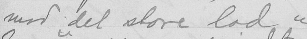mod "det store lod".
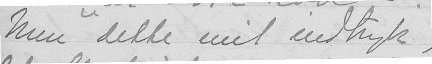Men dette mit indtryk,
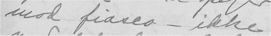mod fiasco - ikke
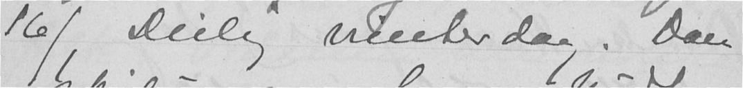16/1 Deilig vinterdag. Don
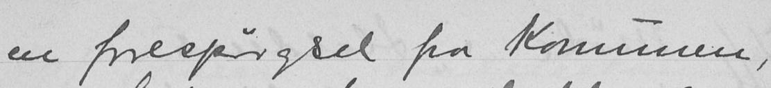en forespørgsel fra komunen,
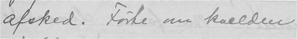afsked. Førte om kvelden
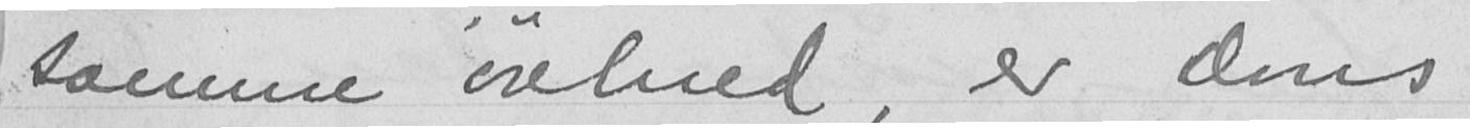samme øiemed, er deres
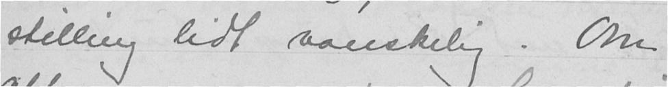stilling lidt vanskelig. Om
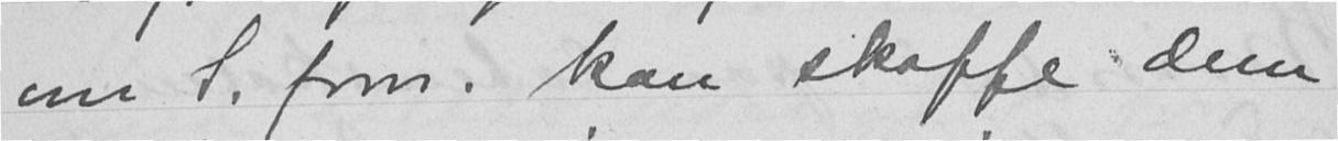om S. forn. kan skaffe dem
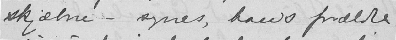skjæbne - synes, hans forældre
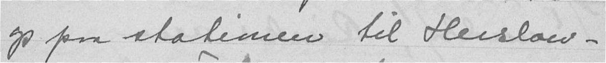op paa stationen til Herslow-
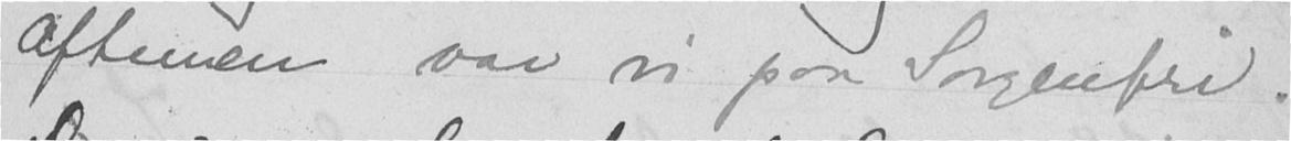aftenen var vi paa Sorgenfri.
